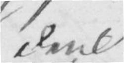den
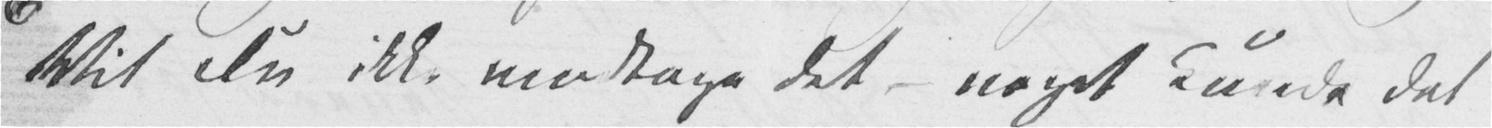Vil Du ikke modtage det – noget kunde det
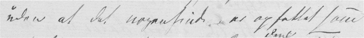uden at det nogensinde er opfattet som
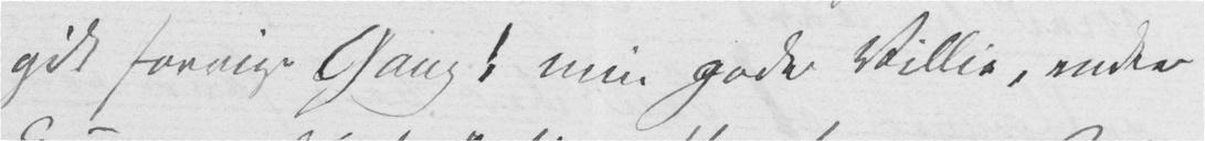gik forrige Gang! min gode Villie, endte
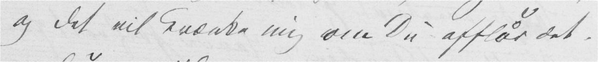og det vil krænke mig om Du afslår det.
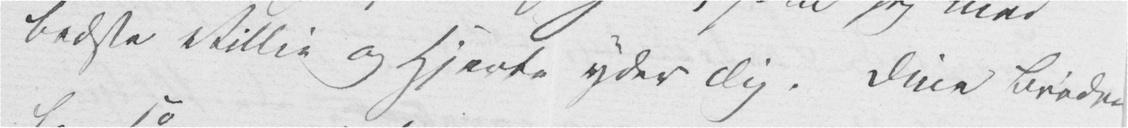bedste Villie og Hjerte yder Dig. Dine Brødre
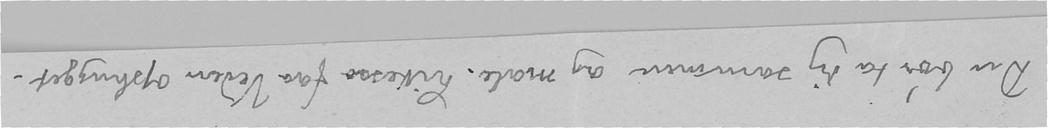Du hør ta dig sammen og male. Likesaa faa Veden ophugget.
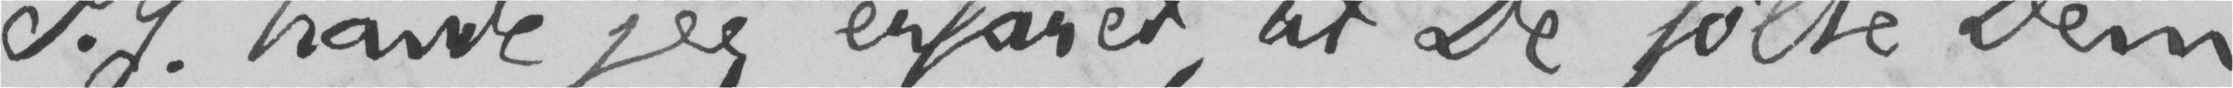S. J. havde jeg erfaret, at De følte Dem
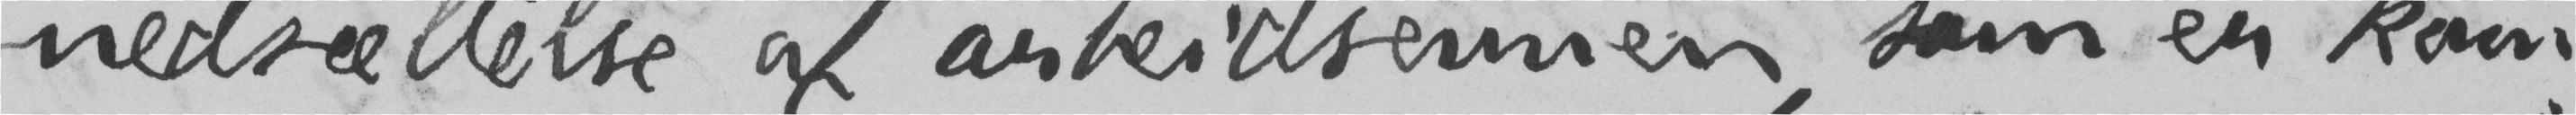nedsættelse af arbeidsevnen, som er kom-
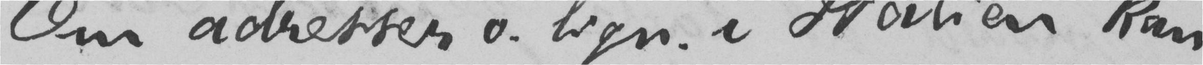Om adresser o.lign. i Italien kan
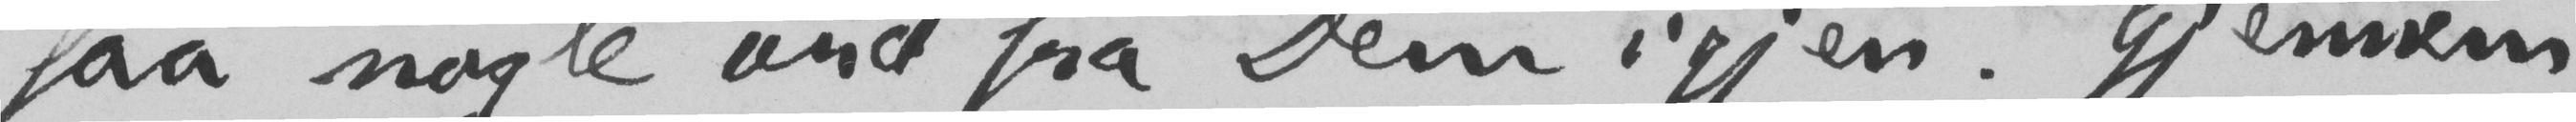faa nogle ord fra Dem igjen. Gjennem
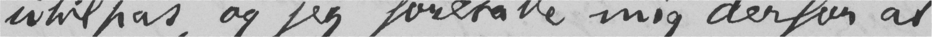utilpas, og jeg foresatte mig derfor at
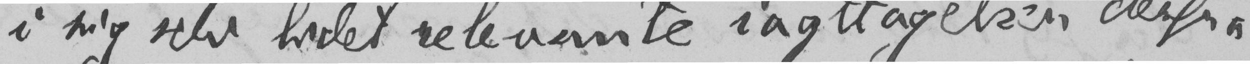i sig selv lidet relevante iagttagelser derfra
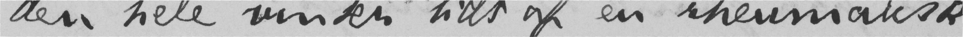den hele vinter lidt af en rheumatisk
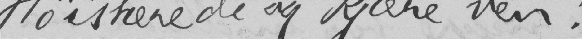Høistærede og kjære ven!
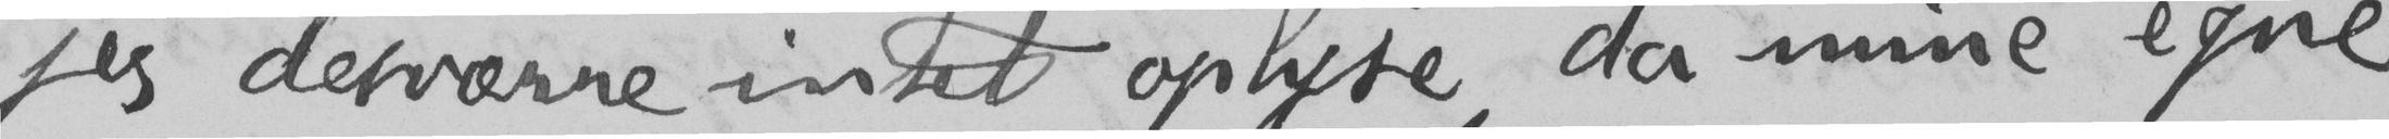jeg desværre intet oplyse, da mine egne
